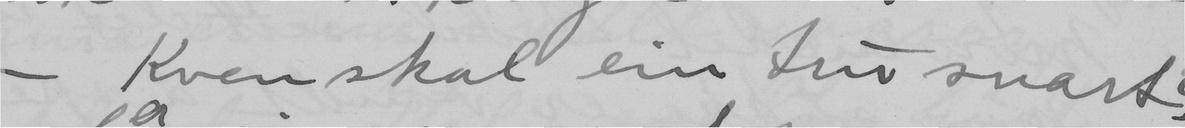Kven skal ein tru snart?
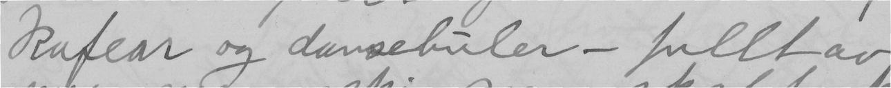kafear og dansebuler – fullt av
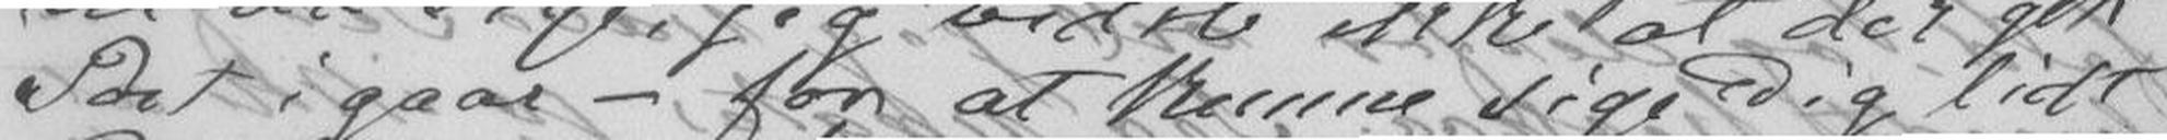Post igaar - for at kunne sige Dig lidt
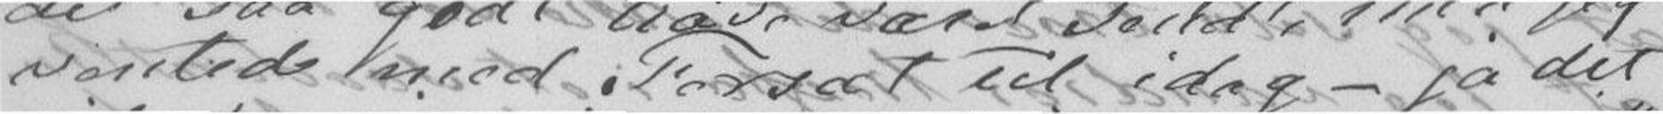ventede med Forsæt til idag - ja det
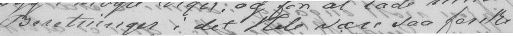Beretninger i det Hele være saa fersk
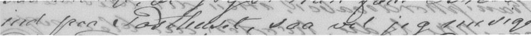ind paa Posthuset, saa vil jeg nu sige
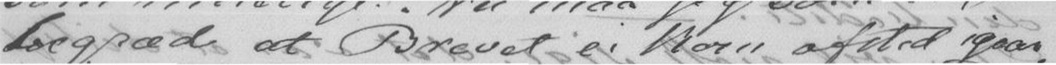Ærgræd at Brevet ei kom afsted igaar,
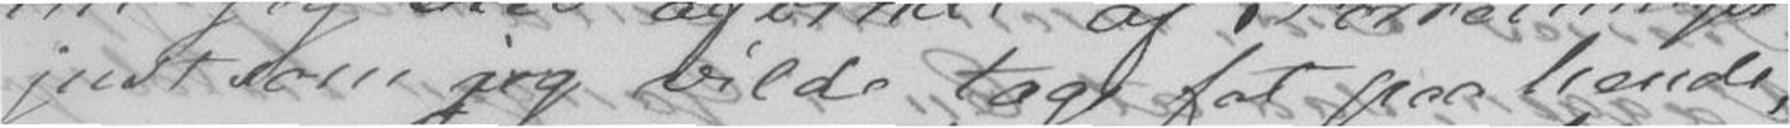just som jeg vilde tage fat paa hende
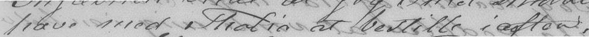have med Tholia at bestille i aften.
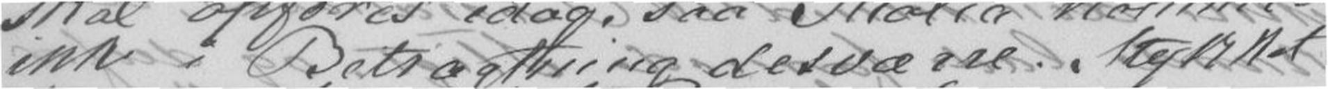ikke i Betragtning desværre. Stykket
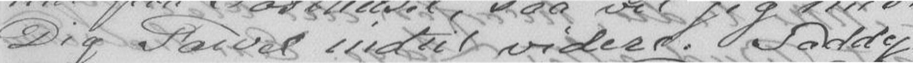Dig Farvel indtil videre. Paddy.
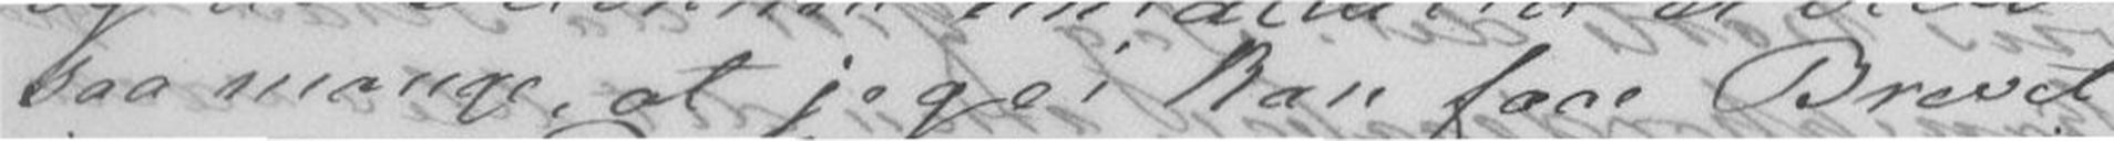saa mange, at jeg ei kan faae Brevet
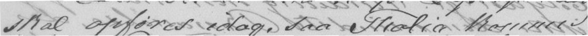skal opføres idag, saa Tholia kommer
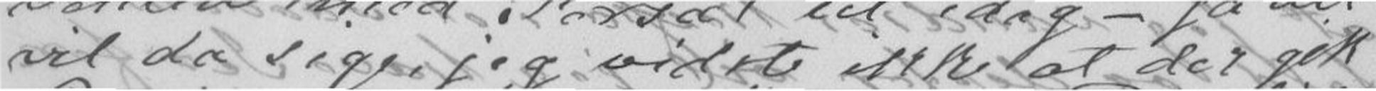vil da sige jeg vidste ikke at der gik
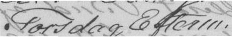Torsdag Efterm.
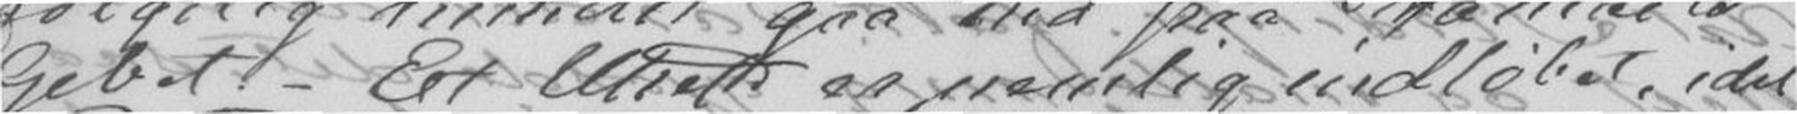Gebet. - Et Uhel er nemlig indløbet, idet
